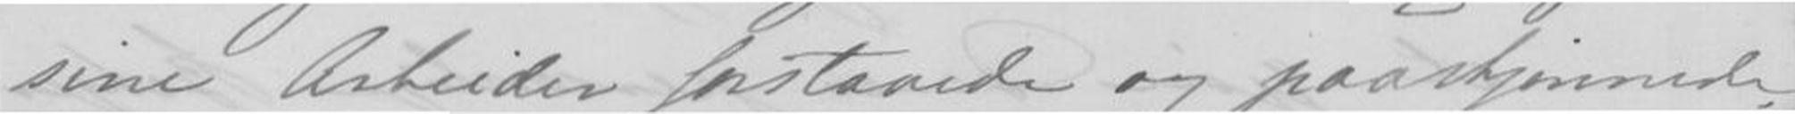sine Arbeider forstaaede og paaskjønnede,
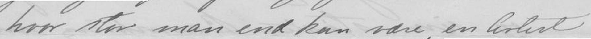hvor stor man end kan være, en Artist
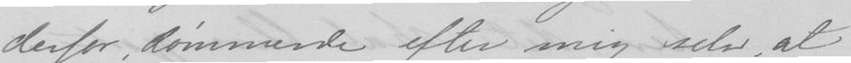derfor dømmende efter mig selv, at
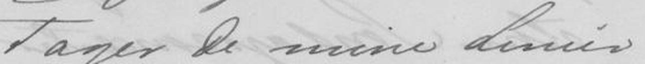Tager De mine Linier
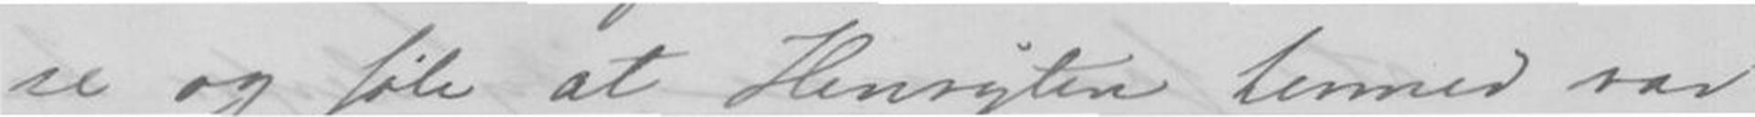se og føle at Hensigten hermed var
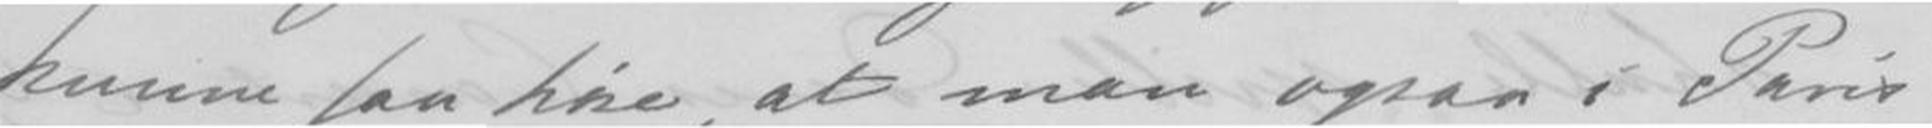kunne faa høre, at man ogsaa i Paris
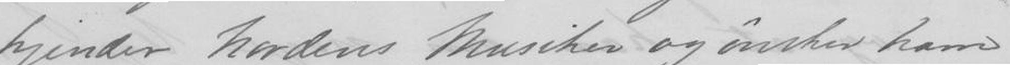kjender Nordens Musiker og ønsker ham
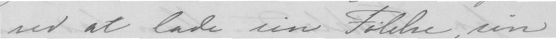ved at lade sin Følelse, sin
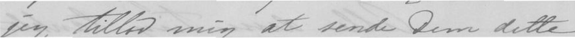jeg tillod mig at sende Dem dette
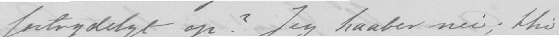fortrydeligt op? Jeg haaber nei; thi
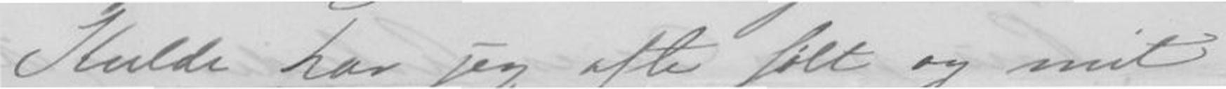Kulde har jeg ofte følt og mit
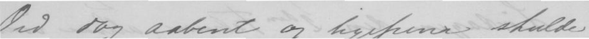Ord dog aabent og ligefrem skulde
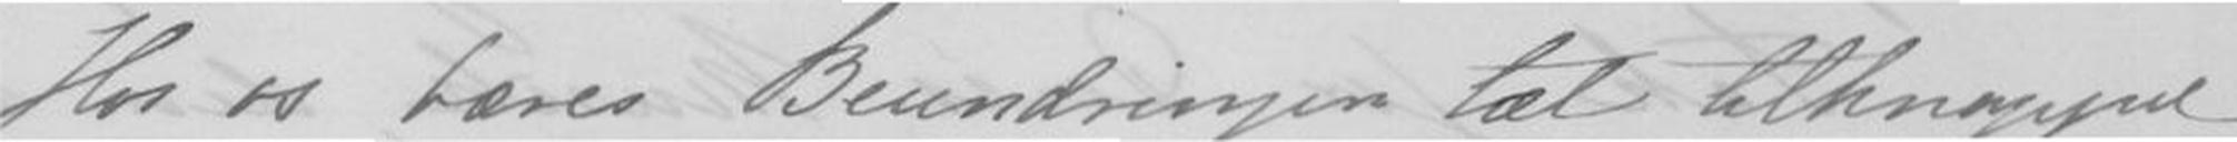Hos os bæres Beundringen tæt tilknappet
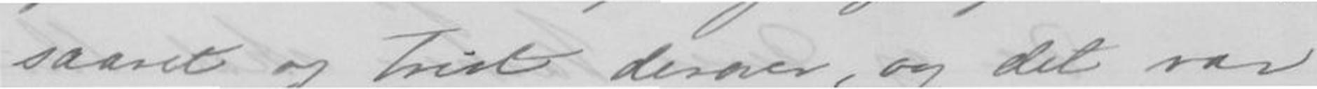saaret og trist derover, og det var
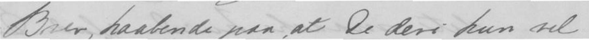Brev, haabende paa, at De deri kun vil
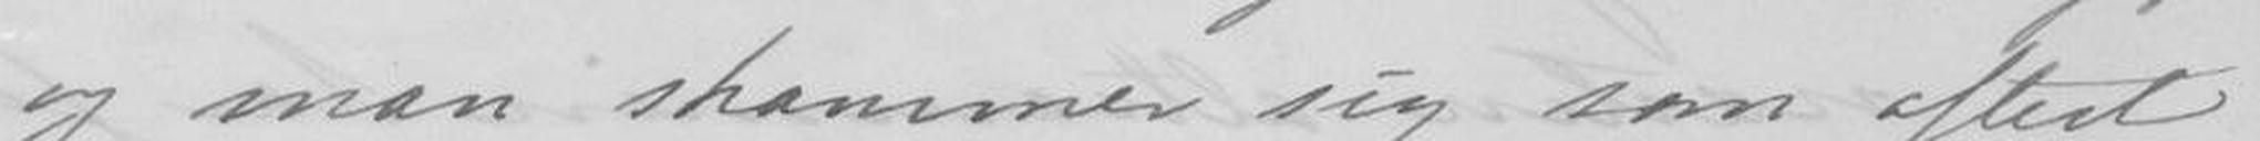og man skammer sig som oftest
